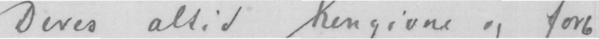Deres altid Hengivne og forb.
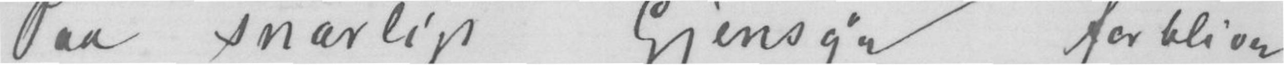Paa snarlig Gjensyn forbliver
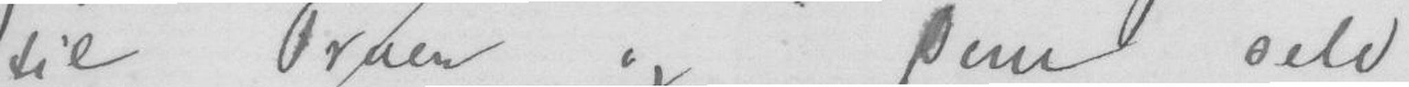til Fruen og Dem selv.
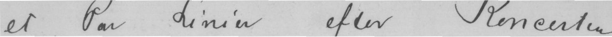et par Linier efter Koncerten
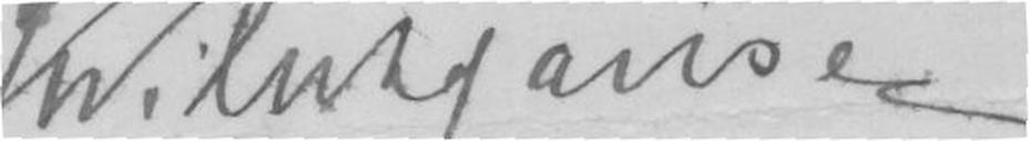Wilhelm Hansen
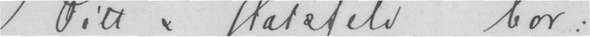Pitt & Hatzfeld bor:
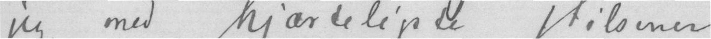jeg med hjærteligste Hilsener
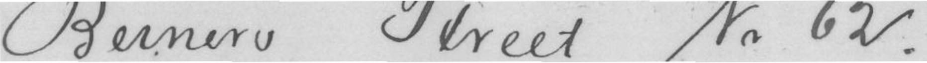Berneru Street No. 62.
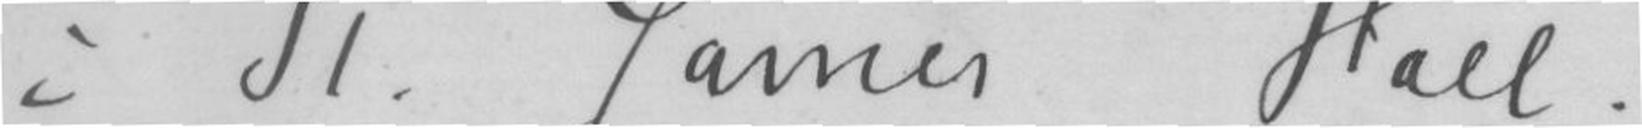i St. Jamer Hall.
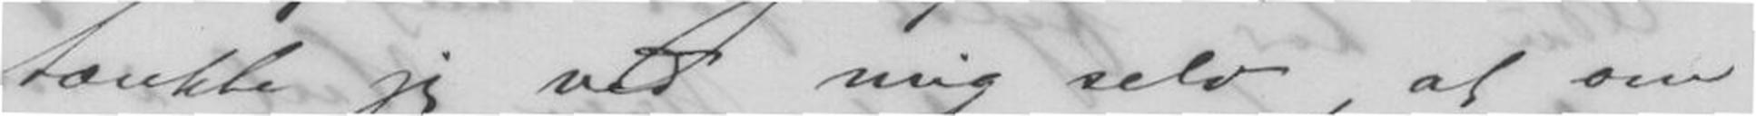tænkte jeg ved mig selv, at om
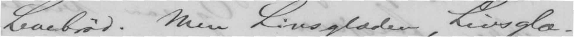Levebrød. Men Livsglæden, Livsglæ-
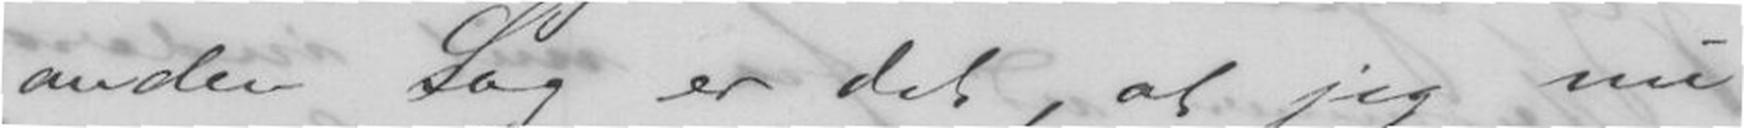anden Sag er det, at jeg nu
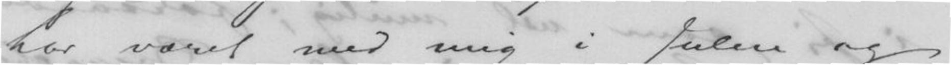har været med mig i Julen og
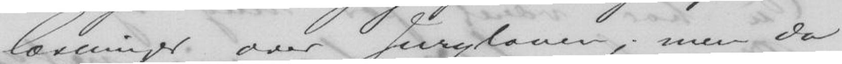læsninger over Juryloven, men da
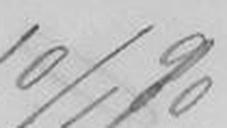10/1 90
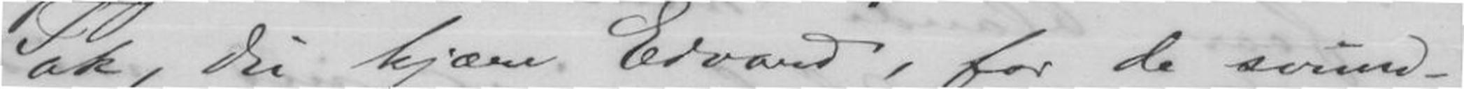Tak, du kjære Edvard, for de svund-
